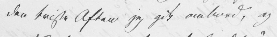den triste Aften jeg gik ombord, og
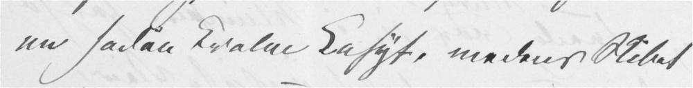en sadan kvalm Kahyt, medens Skibet
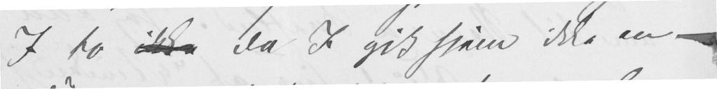I to ikke da I gik hjem ikke en
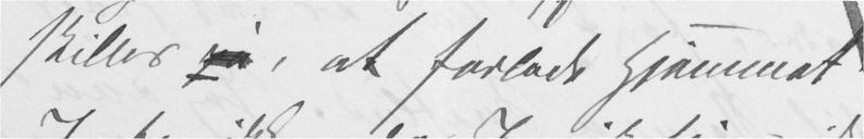skilles på, at forlade Hjemmet
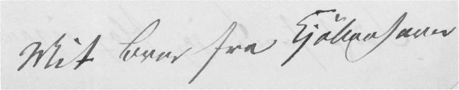Mit Brev fra Kjøbenhavn
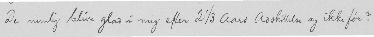De nemlig blive glad i mig efter 2/3 Aars Adskillelse og ikke før?
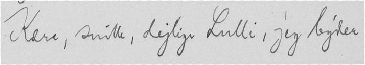Kære, snille, dejlige Lulli, jeg byder
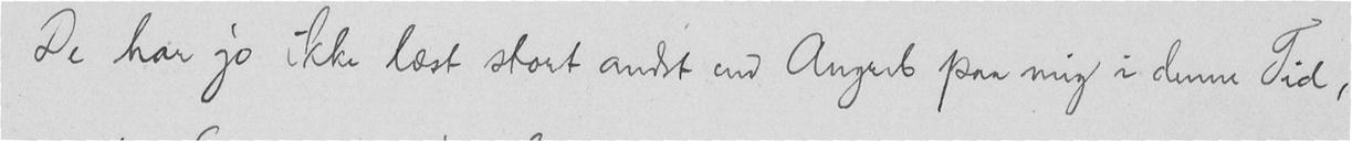De har jo ikke læst stort andet end Angreb paa mig i denne Tid,
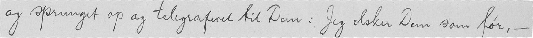og sprunget op og telegraferet til Dem: Jeg elsker Dem som før, -
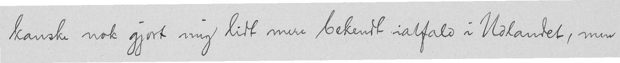kanske nok gjort mig lidt mere bekendt ialfald i Udlandet, men
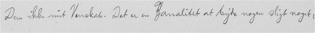Dem ikke mit Venskab. Det er en banalitet at byde nogen sligt noget;
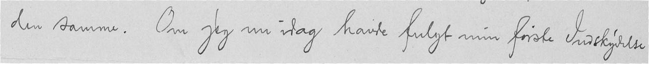den samme. Om jeg nu idag havde fulgt min første Indskydelse
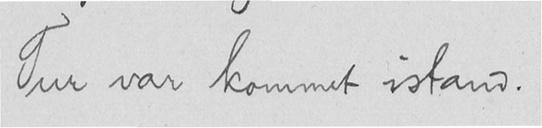Tur var kommet istand.
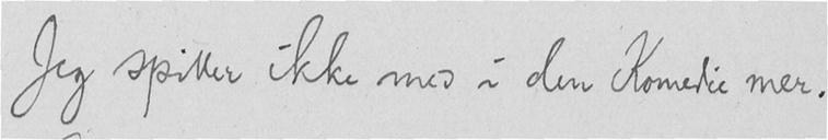Jeg spiller ikke med i den Komedie mer.
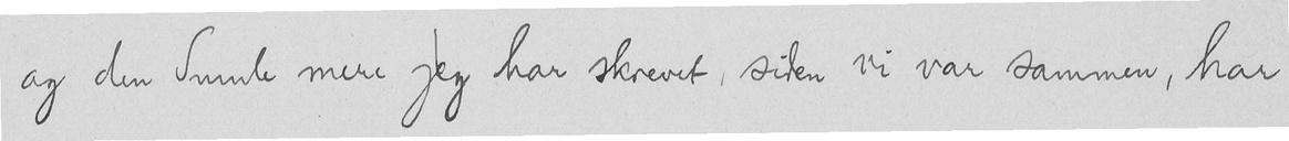og den Smule mere jeg har skrevet, siden vi var sammen, har
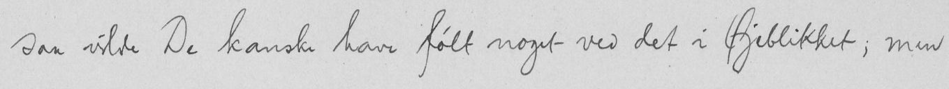saa vilde De kanske have følt noget ved det i Øjeblikket; men
